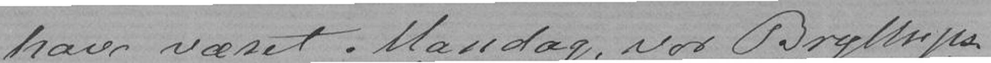have været Mandag, vor Bryllups-
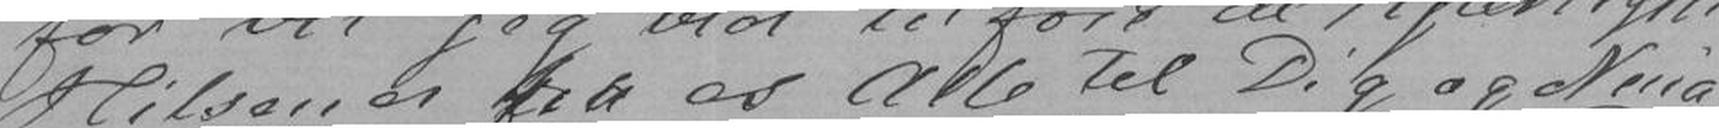Hilsener fra os Alle til Dig og Nina,
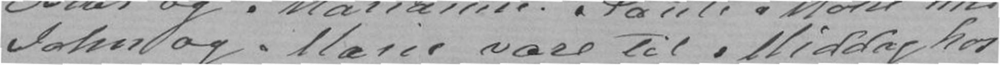John og Marie vare til Middag hos
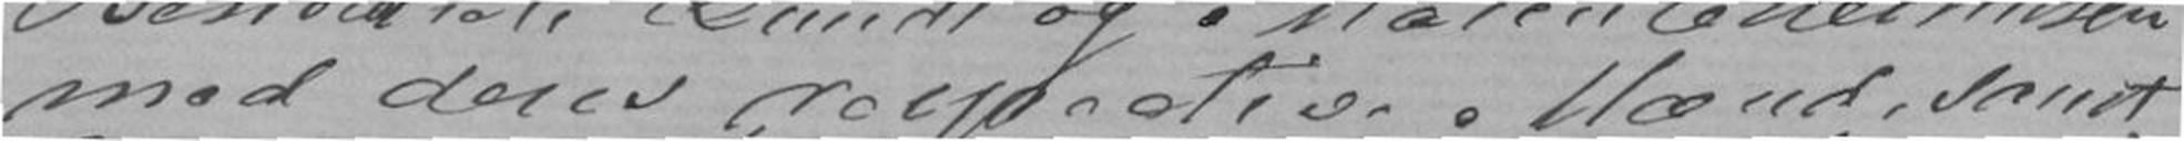med deres respective Mænd, samt
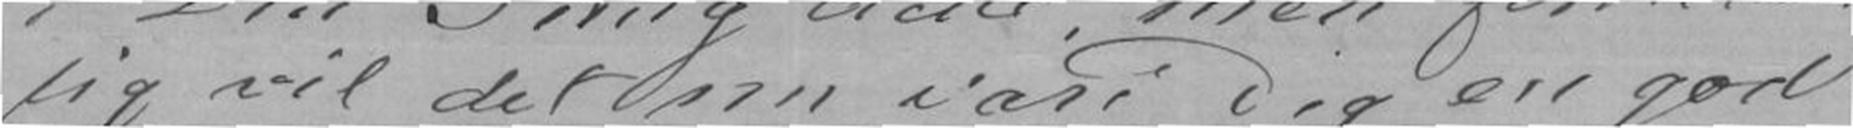lig vil det nu være Dig en god
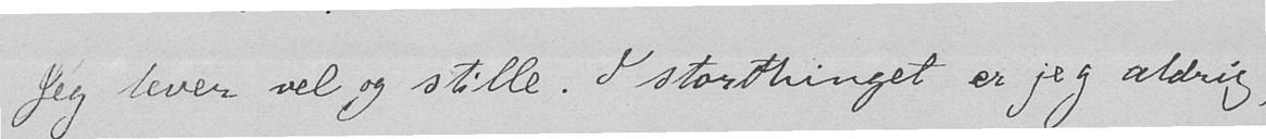Jeg lever vel og stille. I storthinget er jeg aldrig,
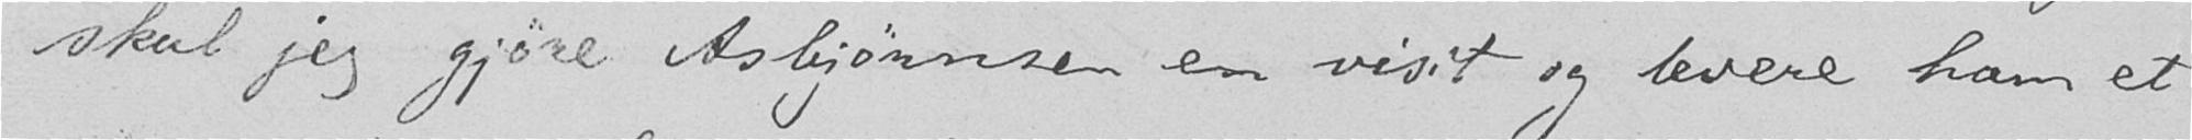skal jeg gjøre Asbjørnsen en visit og levere ham et
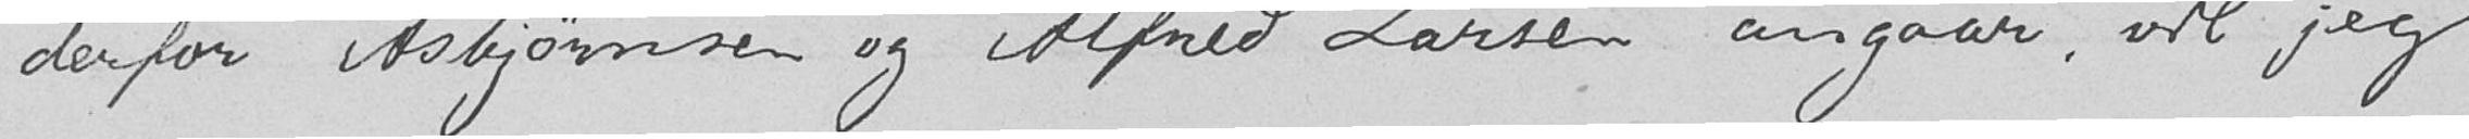derfor Asbjørnsen og Alfred Larsen angaar, vil jeg
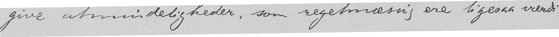give almindeligheder, som regelmæssig ere ligesaa værdi-
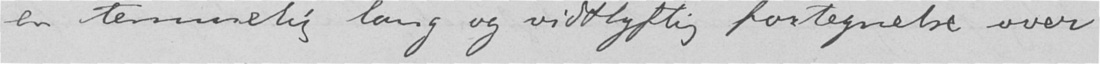en temmelig lang og vidtlyftig fortegnelse over
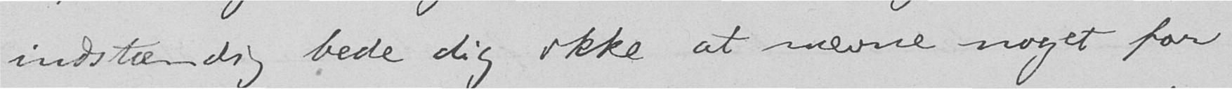indstændig bede dig ikke at nævne noget for
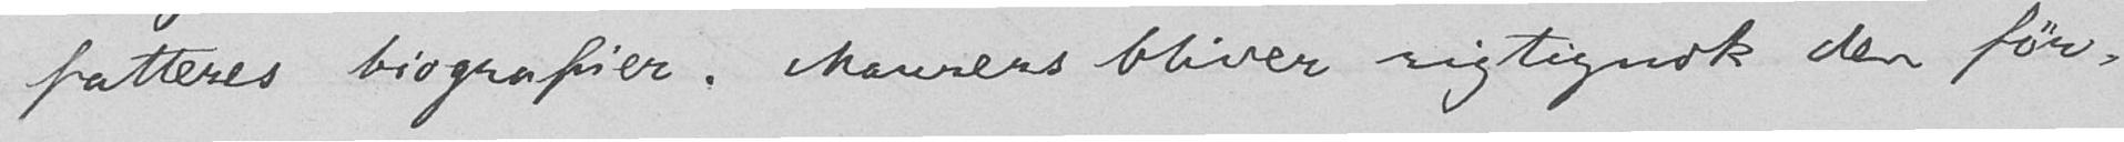fatteres biografier. Maurers bliver rigtignok den før-
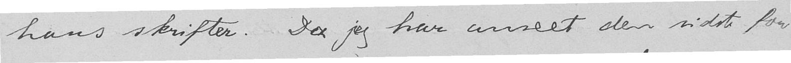hans skrifter. Da jeg har anseet den sidste for
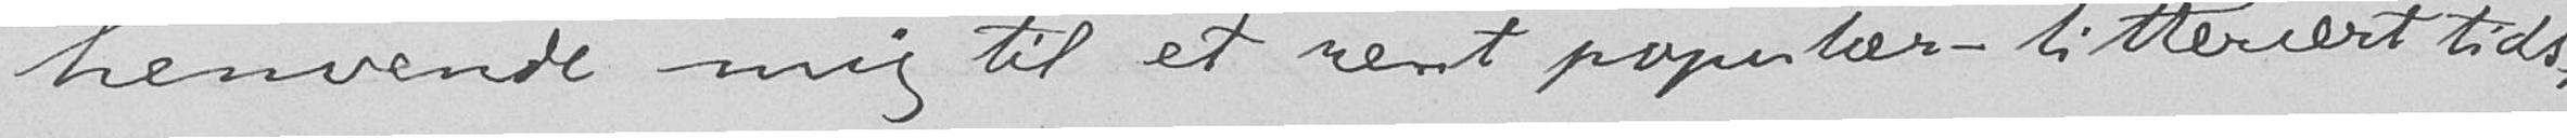henvende mig til et rent populær-litterært tids-
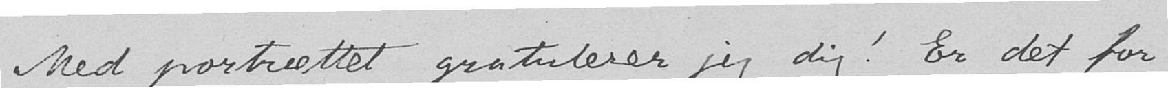Med portrættet gratulerer jeg dig! Er det for
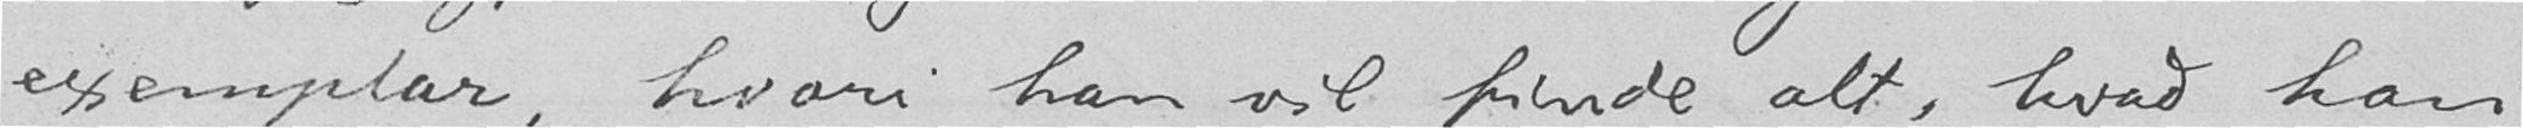exemplar, hvori han vil finde alt, hvad han
